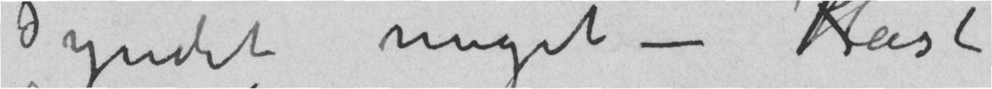syndet meget – Kast
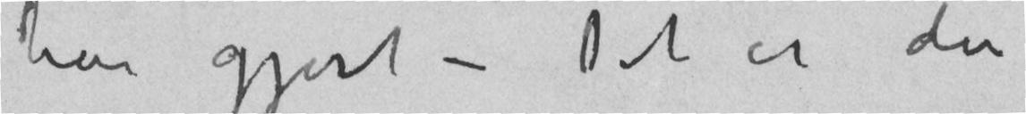har gjort – Det er da
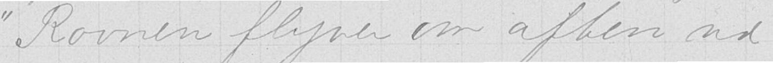"Ravnen flyver om aften ud
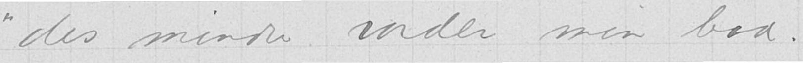"des mindre vorder min bod."
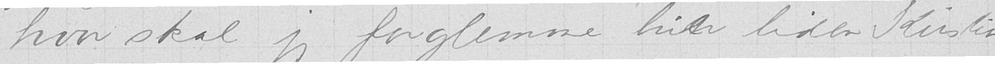"hvor skal jeg forglemme hin liden Kristin.
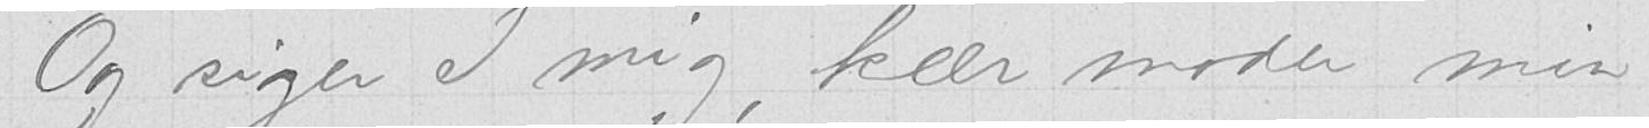"Og siger I mig, kær moder min
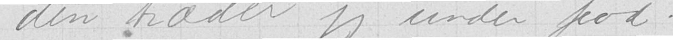"den træder jeg under fod
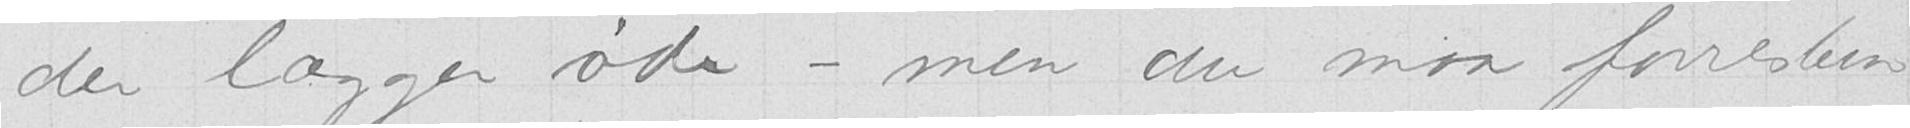der lægger øde — men du maa forresten
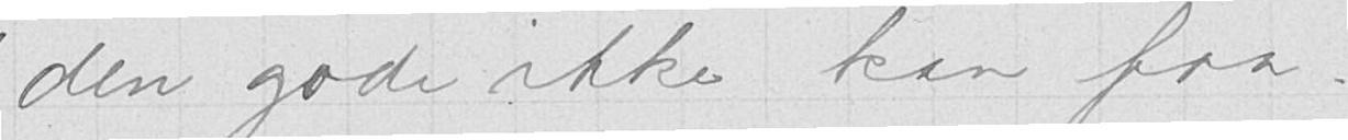"den gode ikke kan faa.
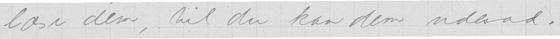læse dem, til du kan dem udenad.
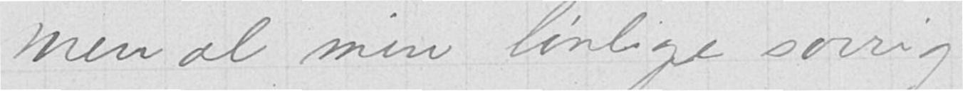"Men al min lønlige sorrig
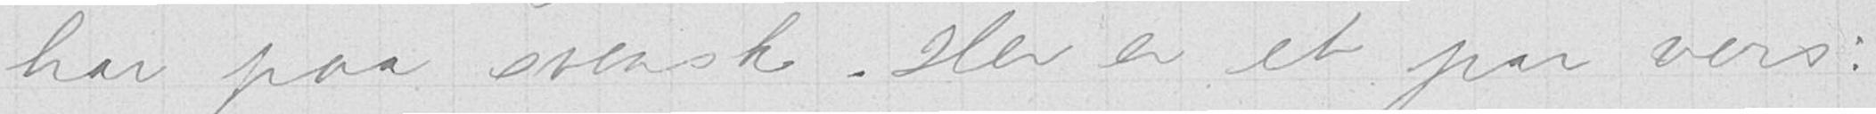har paa svensk. Her er et par vers:
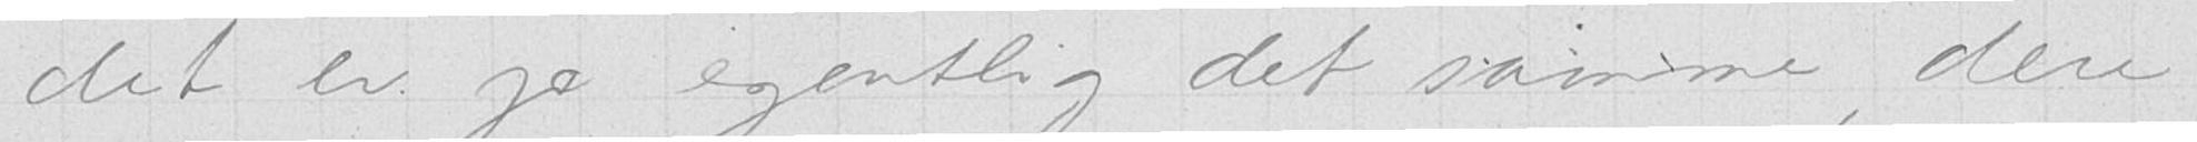Det er jo egentlig det samme, dere
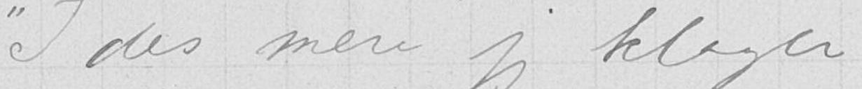"l des mere jeg klager
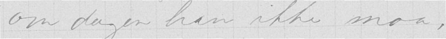"om dagen han ikke maa,
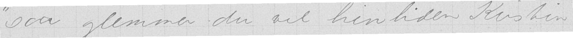"saa glemmer du vel hin liden Kristin
